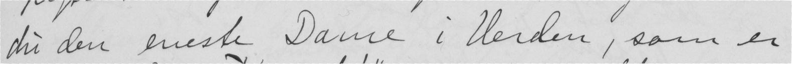Du den eneste Dame i Verden, som er
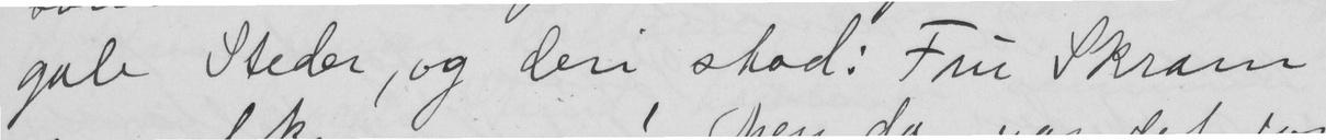gale Steder, og deri stod: Fru Skram
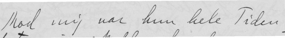Mod mig var hun hele Tiden
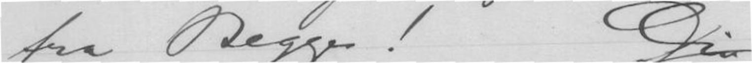fra Begge! Din
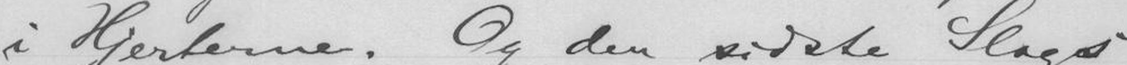i Hjerterne. Og den sidste Slags
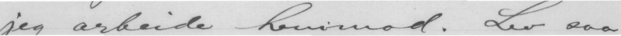jeg arbeide henimod. Lev saa
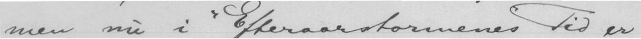men nu i "Efteraarstormens" Tid er
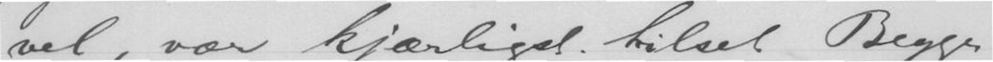vel, vær kjærligst hilset Begge
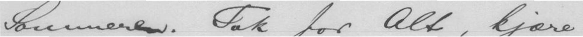Sommeren. Tak for Alt, kjære
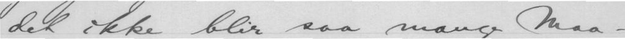det ikke blir saa mange Maa-
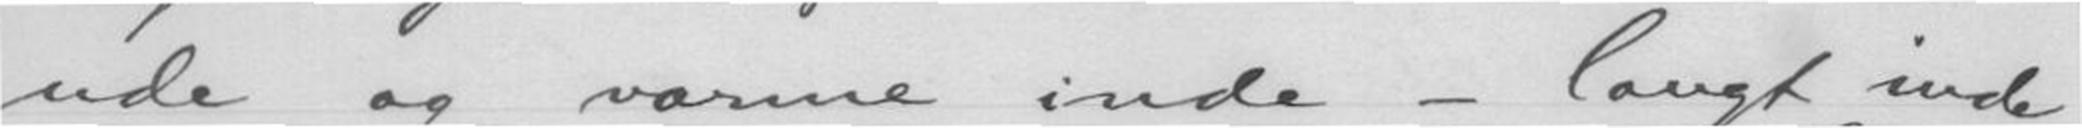ude og varme inde - langt inde
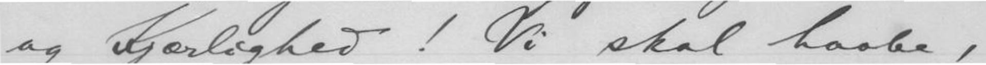og Kjærlighed! Vi skal haabe,
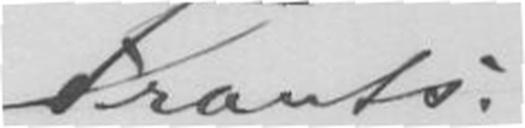Frants.
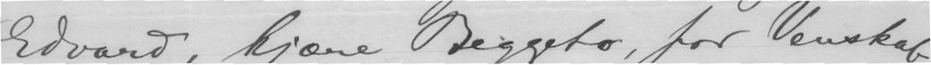Edvard, kjære Beggeto, for Venskab
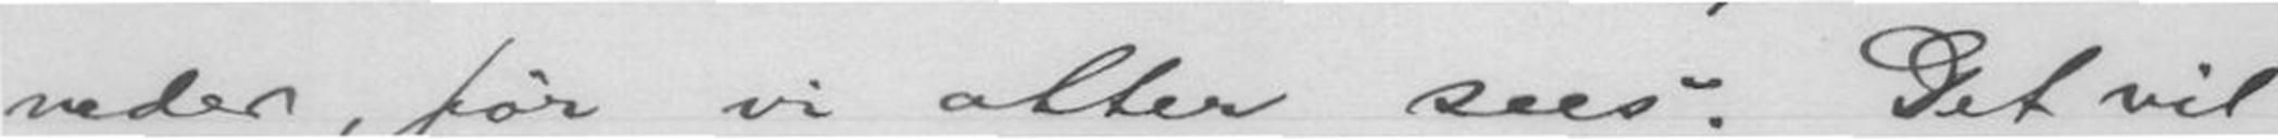neder, før vi atter sees. Det vil
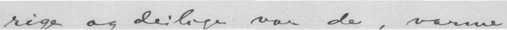rige og deilige var de, varme
